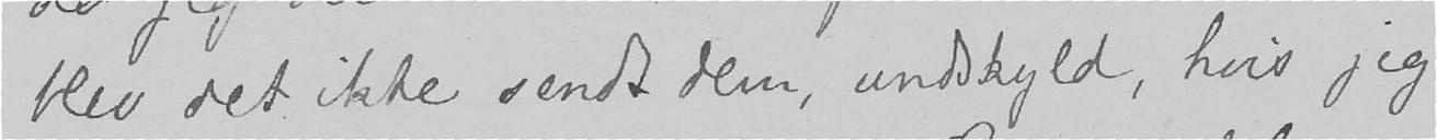blev det ikke sendt dem, undskyld, hvis jeg
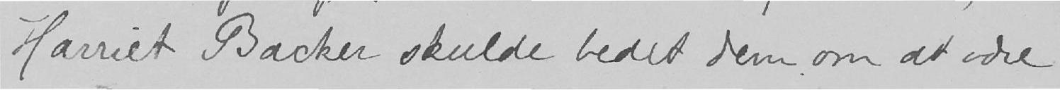Harriet Backer skulde bedet dem om at være
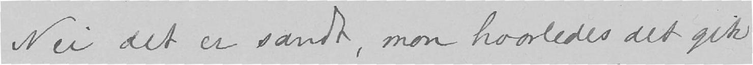Nei det er sandt, mon hvorledes det gik
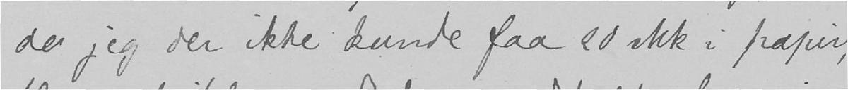da jeg der ikke kunde faa 20 Mk i papir,
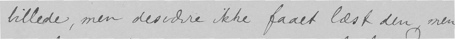billede, men desværre ikke faaet læst den, men
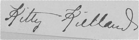Kitty Kielland
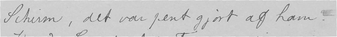Schirm, det var pent gjort af ham.?
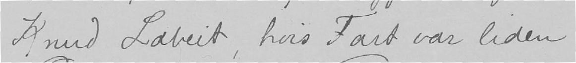Knud Labeit, hvis Fart var liden
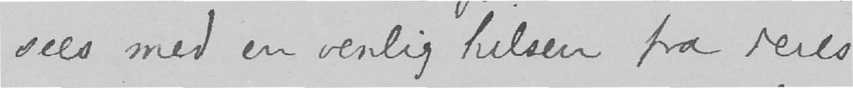sees med en venlig hilsen fra deres
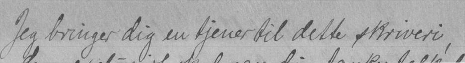Jeg bringer dig en tjener til dette skriveri,
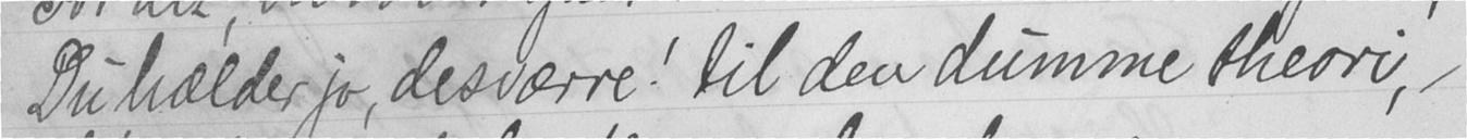Du hælder jo, desværre! til den dumme theori, -
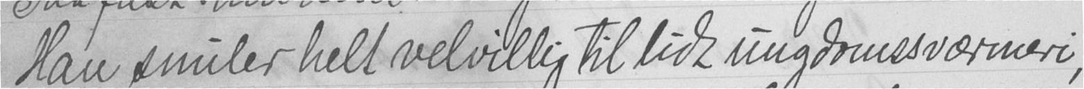Han smiler helt velvillig til lidt ungdomssværmeri,
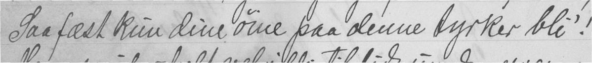Saa fæst kun dine øine paa denne tyrker bli'!
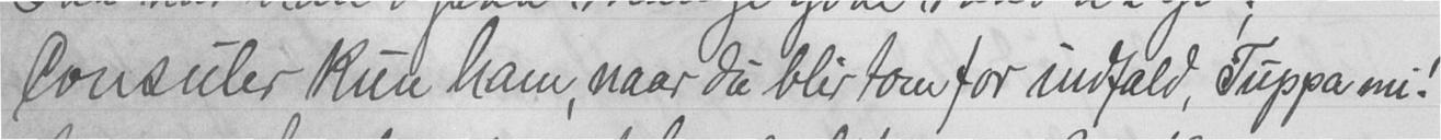Consuler kun ham, naar du blir tom for indfald, Tuppa mi!
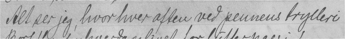Alt ser jeg, hvor hver aften ved pennens trylleri
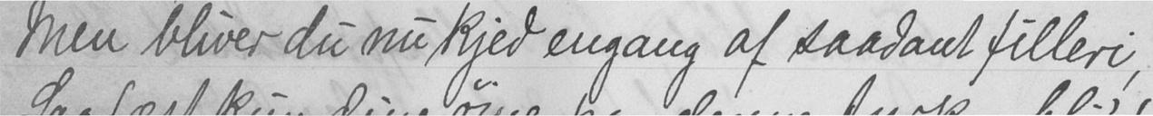Men bliver du nu kjed engang af saadant filleri,
